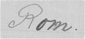Rom.
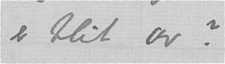er blit av?
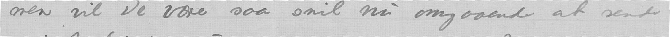men vil De være saa snil nu omgaaende at sende
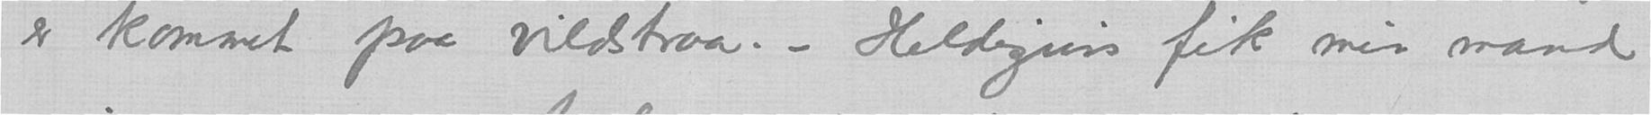er kommet paa vildstraa. – Heldigvis fik min mand
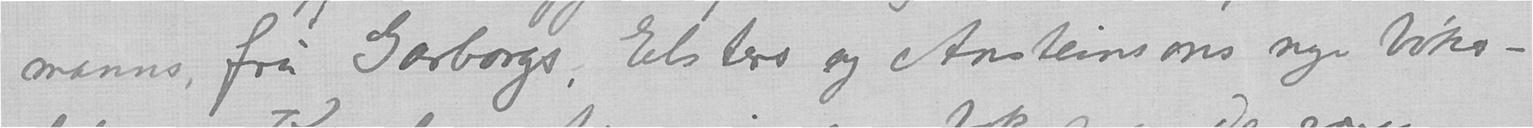manns, fru Garborgs, Elsters og Ansteinsons nye bøker -
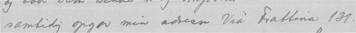samtidig opgav min adresse Via Frattina 138.
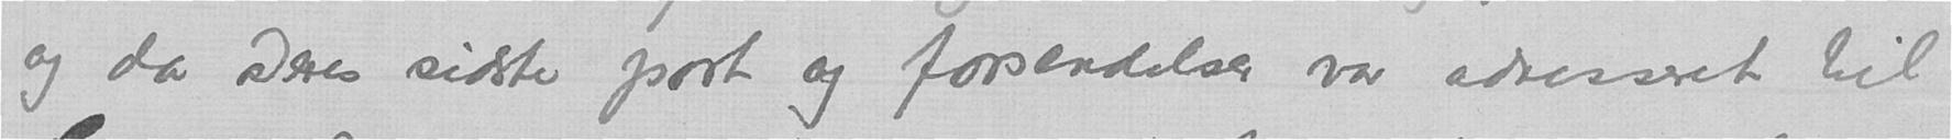og da Deres sidste post og forsendelser var adresseret til
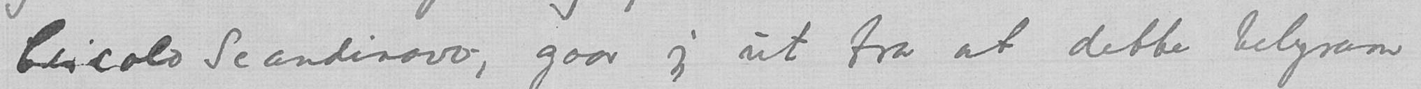Circolo Scandinavo, gaar jeg ut fra at dette telegram
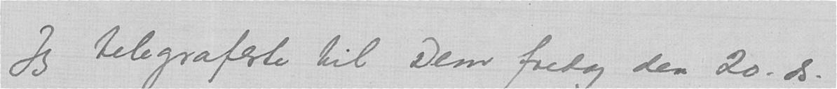Jeg telegraferte til Dem fredag den 20.ds.
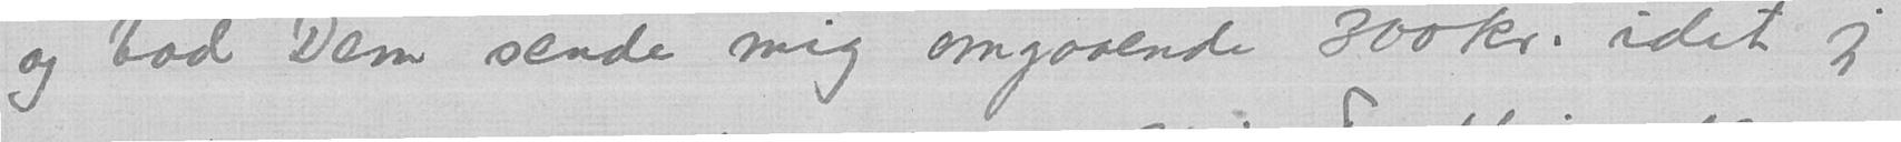og bad Dem sende mig omgaaende 300kr. idet jeg
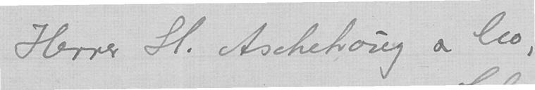Herrer H. Aschehoug & Co,
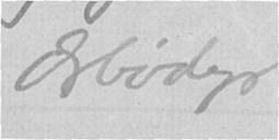Ærbødigst
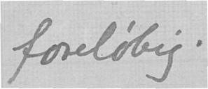foreløbig.
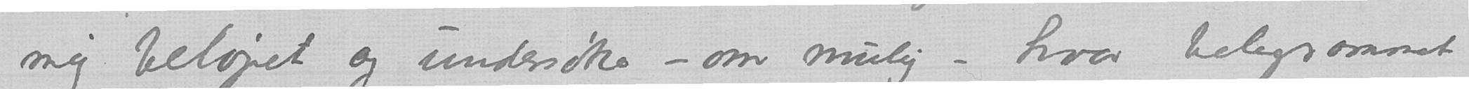mig beløpet og undersøke – om mulig – hvor telegrammet
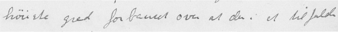høieste grad forbauset over at der i et tilfælde
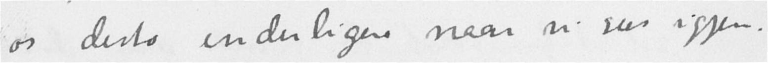os desto inderligere naar vi sees igjen.
